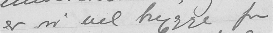er vi vel trygge for
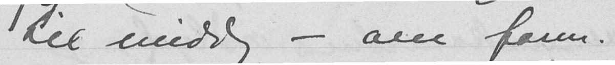til middag - om form.
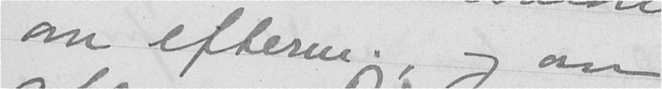om efterm., og om
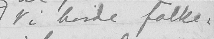Vi havde folke-
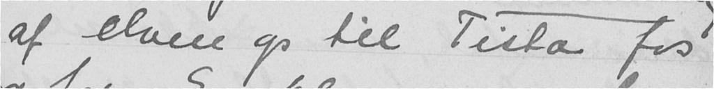af elven op til Tista fos
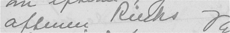aftenen Riecks og
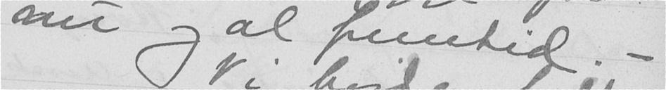nu og al fremtid. -
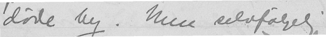døde by. Men selvfølgelig
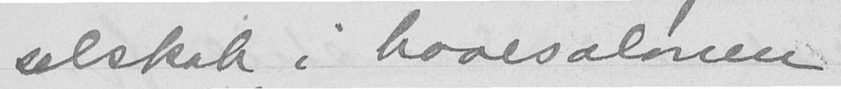selskab i havesalonen
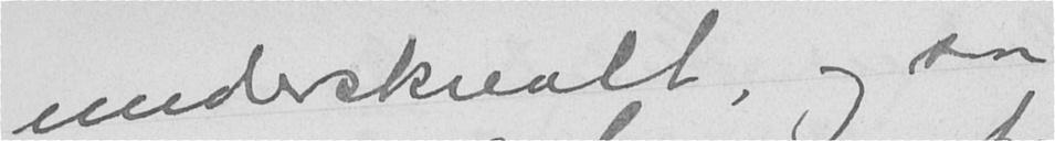underskrevet, og saa
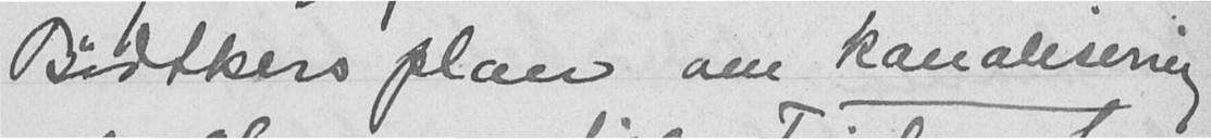Bødtkers plan om kanalisering
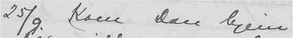25/9 Kom Don hjem
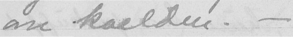om kvelden. -
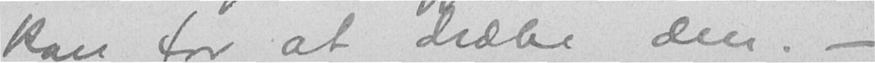kan for at dræbe den. -
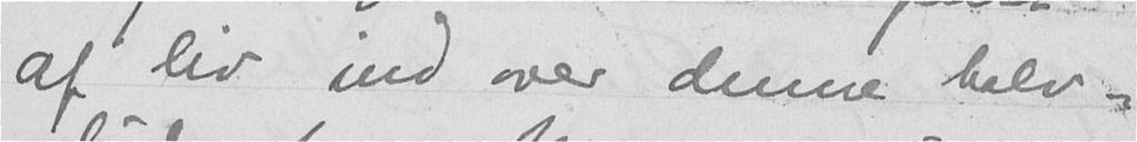af liv ind over denne halv-
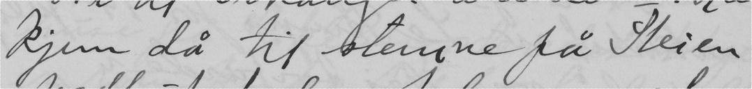kjem då til stemne på Steien
